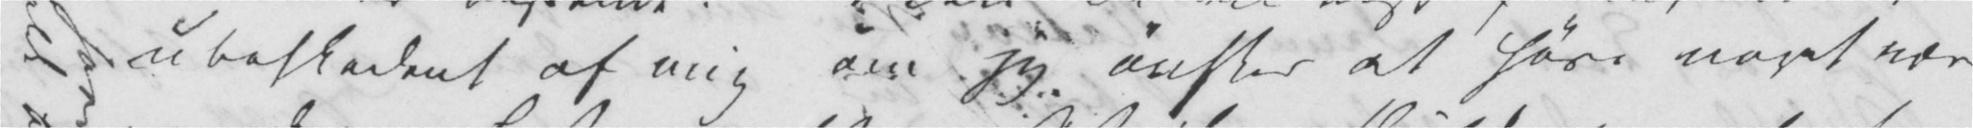ubeskedent af mig om jeg ønsker at høre noget nærmere
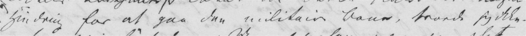Hindring for at gaa den militaire Bane, troede jeg ikke –
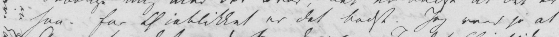saa – for Øieblikket er det bedst. Jeg veed jo at
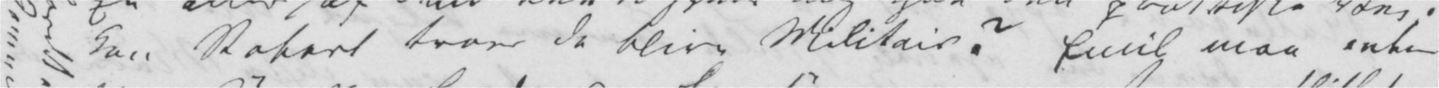Kan Robert troer De blive Militair? Emil maa enten
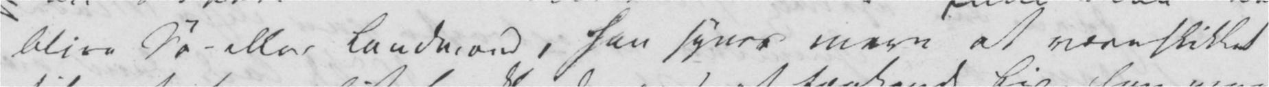blive Sø- eller Landmand, han synes mere at være skikket
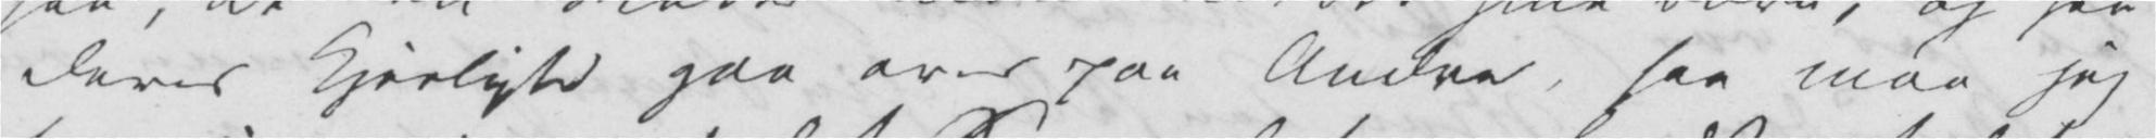deres Kjerlighed gaa over paa Andre, saa maa jeg
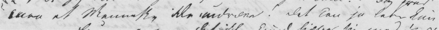maa et Menneske ikke undvære! Det kan jo leve kun
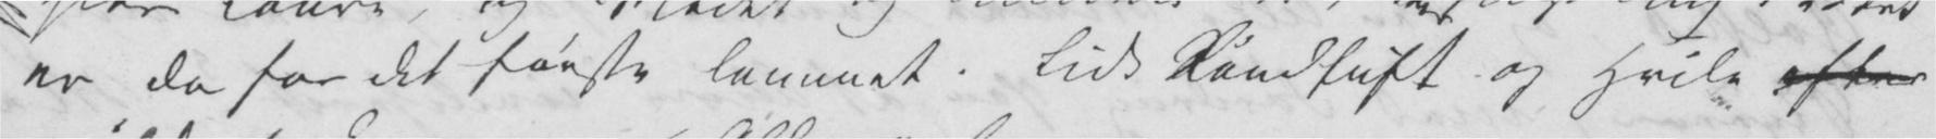er da for det første lammet. Lidt Landluft og Hvile efter
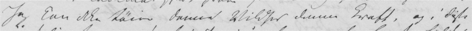Jeg kan ikke tøile denne Vildhed denne Kraft,og i disse
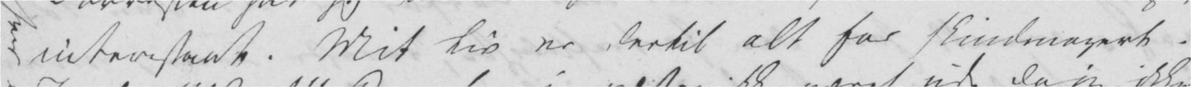interessant. Mit Liv er dertil altfor skindmagert.
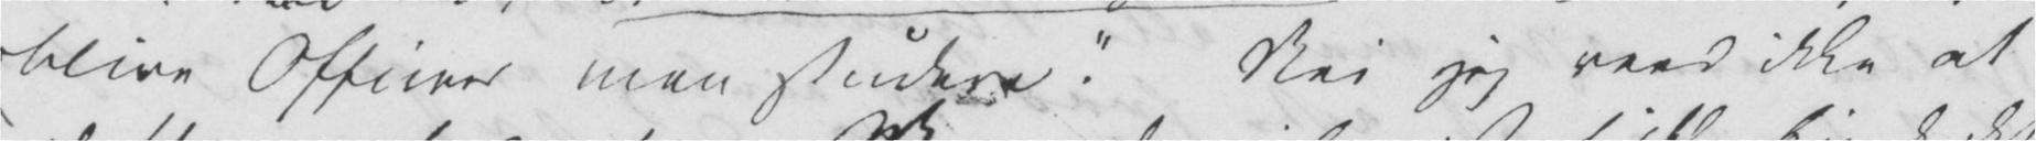blive Officeer men studere.» Nei jeg veed ikke at
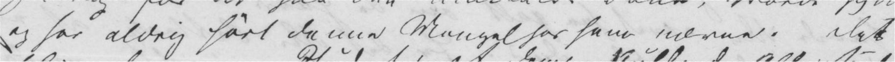og har aldrig hørt denne Mangel hos ham nævne. Det
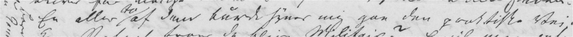En eller to af dem burde synes mig gaa den praktiske Vei.
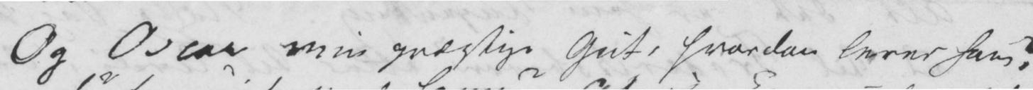Og Oscar min prægtige Gut, hvordan lever han?
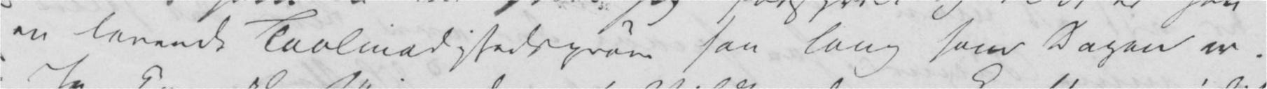en levende Taalmodighedsprøve saa lang som Dagen er.
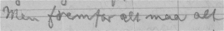Men fremfor alt maa alt
